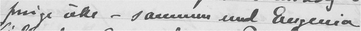forrige uke - sammen med Eugenia
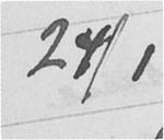24/1
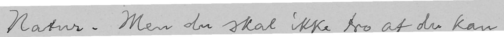Natur. Men du skal ikke tro at du kan
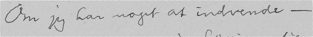Om jeg har noget at indvende -
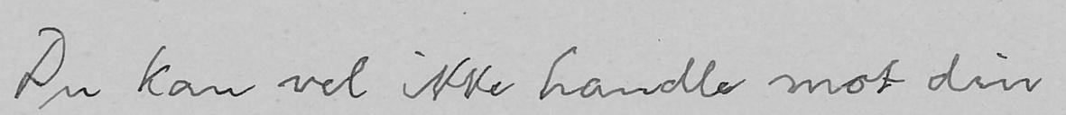Du kan vel ikke handle mot din
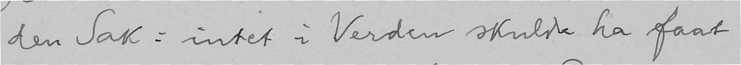den Sak: intet i Verden skulde ha faat
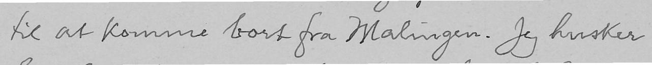til at komme bort fra Malingen. Jeg husker
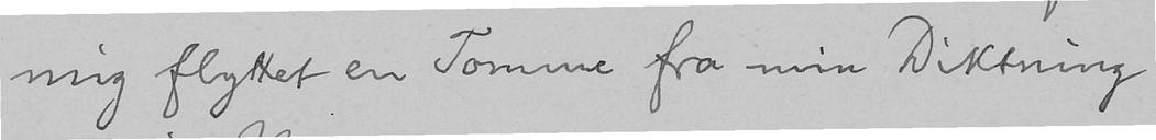mig flyttet en Tomme fra min Diktning
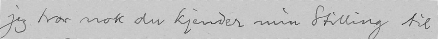jeg tror nok du kjender min Stilling til
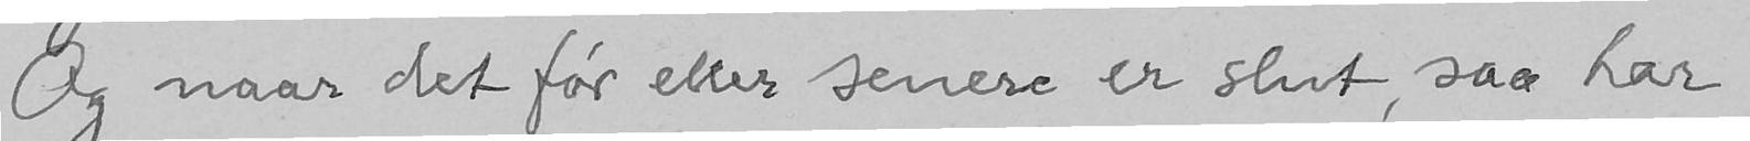Og naar det før eller senere er slut, saa har
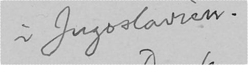i Jugoslavien.
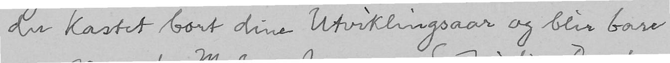du kastet bort dine Utviklingsaar og blir bare
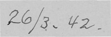26/3. 42.
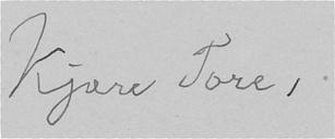Kjære Tore,
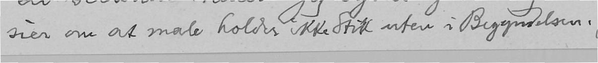sier om at male holder ikke Stik uten i Begyndelsen.
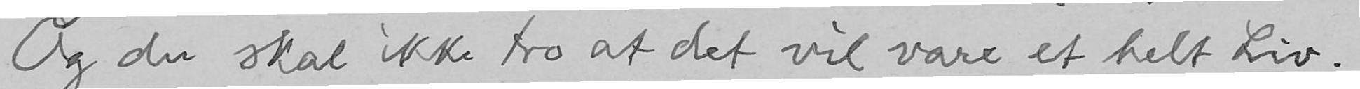Og du skal ikke tro at det vil vare et helt Liv.
